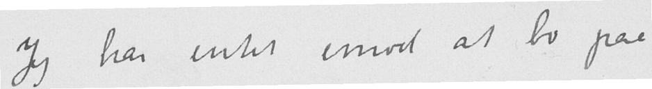Jeg har intet imod at bo paa
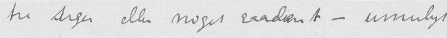tre uger eller noget saadant - umuligt
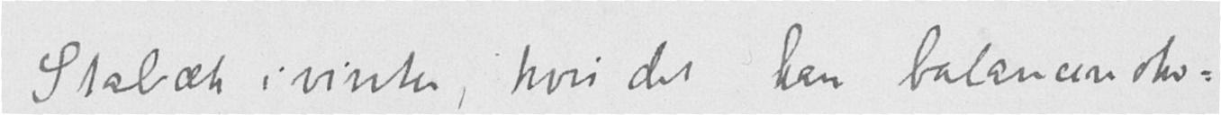Stabæk i vinter, hvis det kan balancere øko-
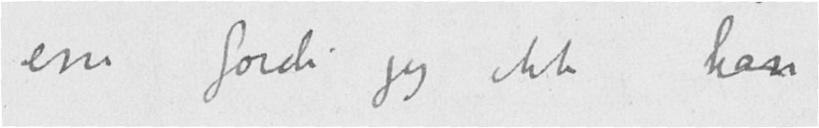ene fordi jeg ikke kan
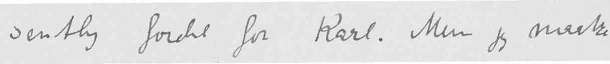sentlig fordel for Karl. Men jeg maatte
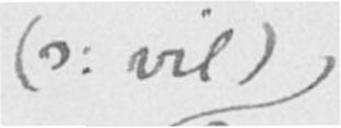(s: vil)
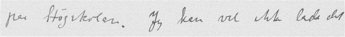paa Høgskolan. Jeg kan vel ikke lade det
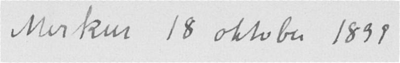Merkur 18 oktober 1899
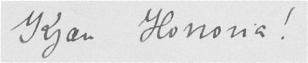Kjære Honoria!
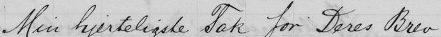Min hjerteligste Tak for Deres Brev
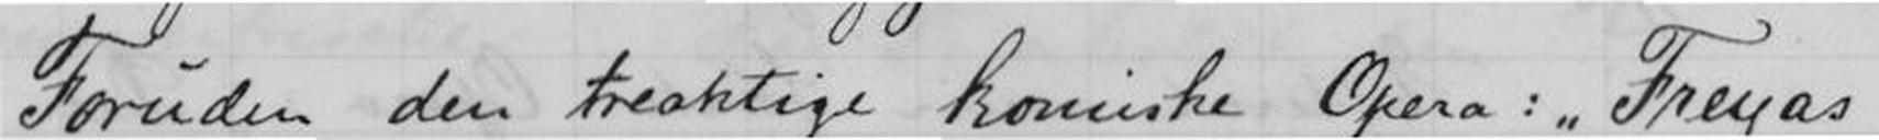Foruden den treaktige komiske Opera "Freyas
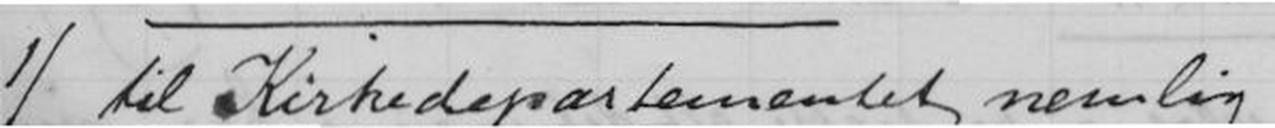1/ til Kirkedepartementet nemlig. -
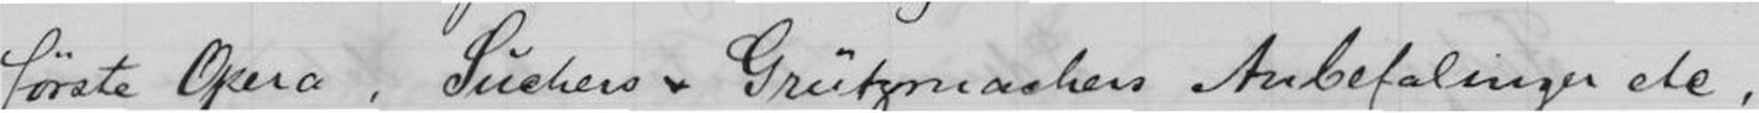første Opera. Suchers & Grützmarchers Anbefalinger etc,
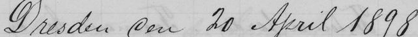Dresden den 20 April 1898
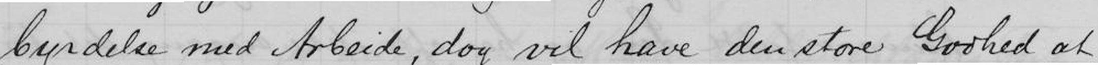byrdelse med Arbeide, dog vil have den store Godhed at
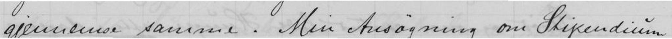gjennemse samme. Min Ansøgning om Stipendium
